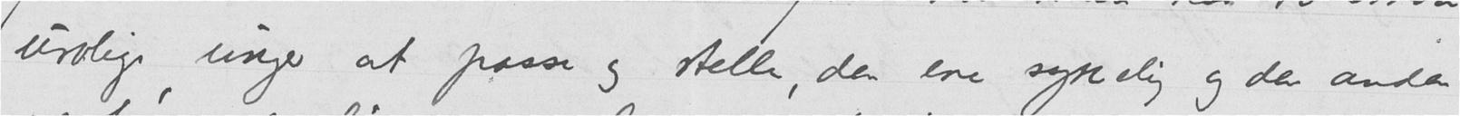urolige unger at passe og stelle, den ene sykelig og den anden
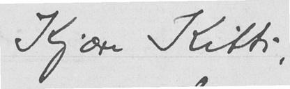Kjære Kitti,
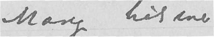Mange hilsener
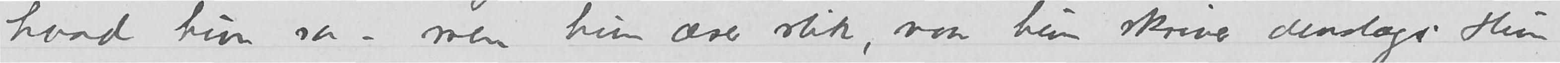hvad hun sa -men hun æser slik, naar hun skriver denslags.
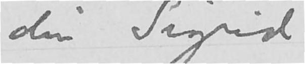din Sigrid
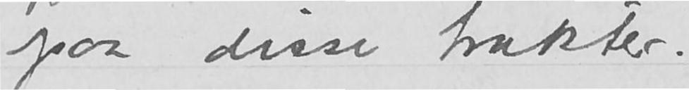paa disse trakter.
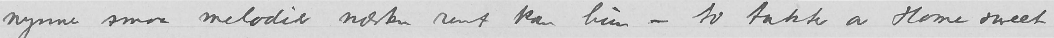nynne smaa melodier næsten rent kan hun – to takter av Home sweet
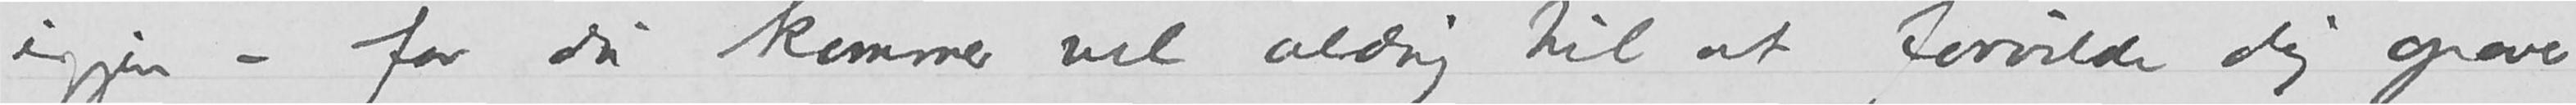igjen -for du kommer vel aldrig til at forvilde dig opover
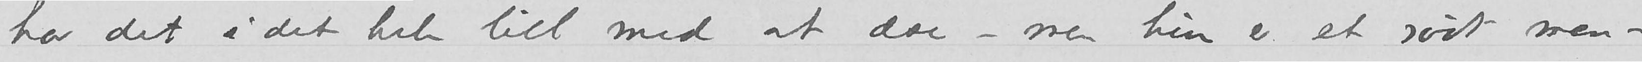Hun har det i det hele litt med at æse -men hun er et sødt men
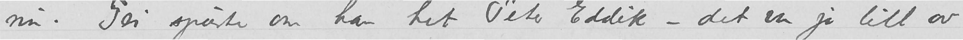siden nu. Pei spurte om han het Peter Eddik -det er jo litt av
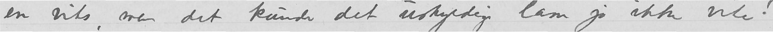en vits, men det kunde det uskyldige lam jo ikke vite!
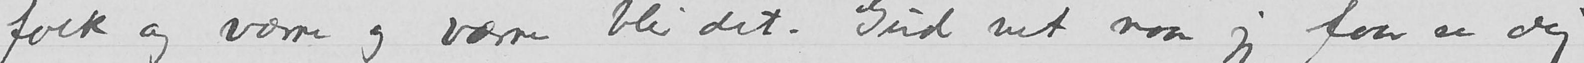folk og væerre og væerre blir det. Gud vet naar jeg faar se dig
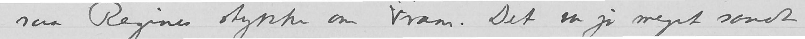Jeg saa Regines stykke om Fram. Det er jo meget sandt
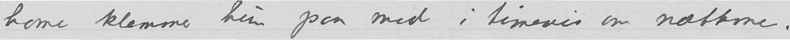home klemmer hun paa med i timevis om nætterne.
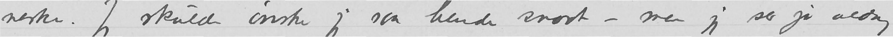neske. Jeg skulle ønske jeg saa hende snart -men jeg ser jo aldrig
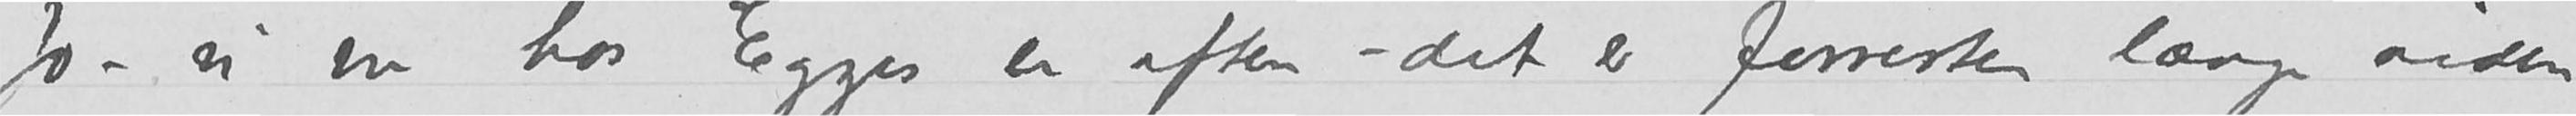Jo, vi var hos Egges en aften -det er forresten længe
